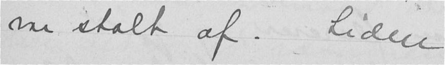var stolt af. Siden
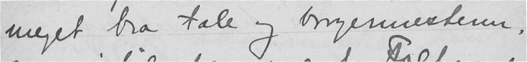meget bra tale og borgermesteren,
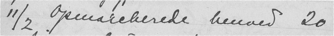11/2 Opmarcherede henved 20
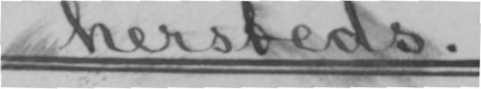hersteds.
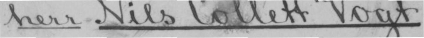herr Nils Collett Vogt.
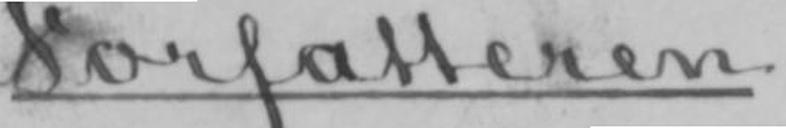Forfatteren
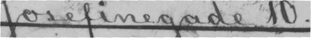Josefinegade 10.
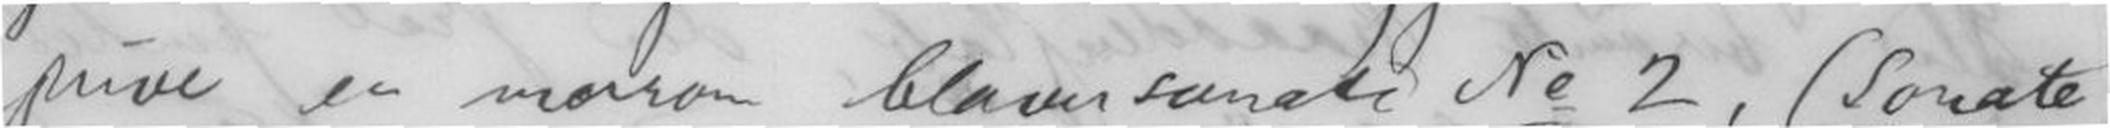skrive en morsom Claversonate No 2, (sonate
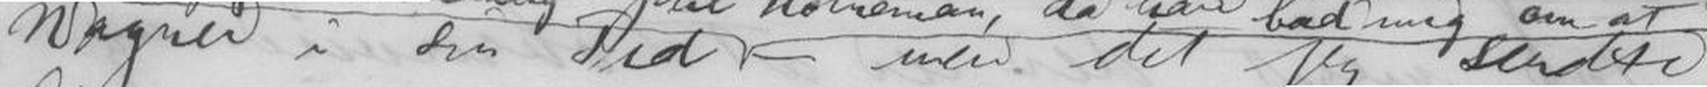Wagner i den Tid - men det jeg sendte
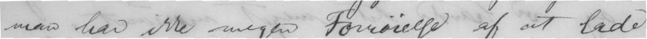man har ikke megen Fornøielse af at lade
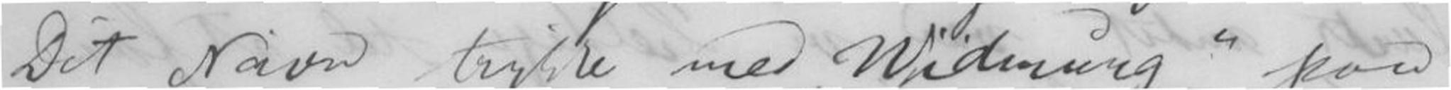Dit Navn trykke med Widmung paa
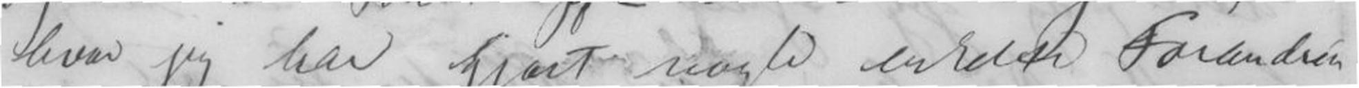hvad jeg har gjort nogle enkelte Forandrin-
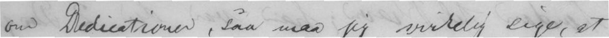om Dedicationen, saa maa jeg virkelig sige, at
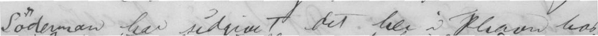Söderman har udgivet det her i Khavn hos
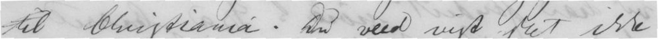til Christiania. Du veed vist slet ikke,
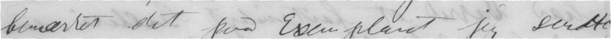bemærket det paa Exemplaret jeg sendte
